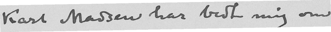Karl Madsen har bedt mig om
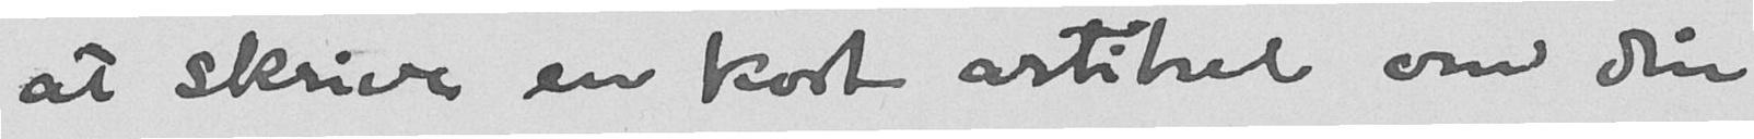at skrive en kort artikel om din
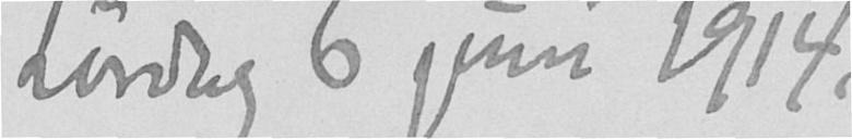Lørdag 6 juni 1914.
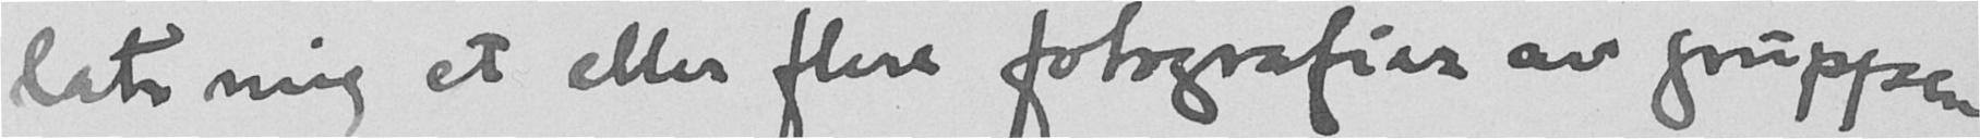late mig et eller flere fotografier av gruppen
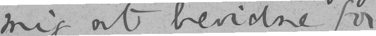mig at bevidne for
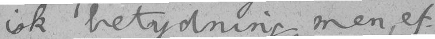iak betydning, men, ef-
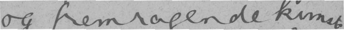og fremragende kunst-
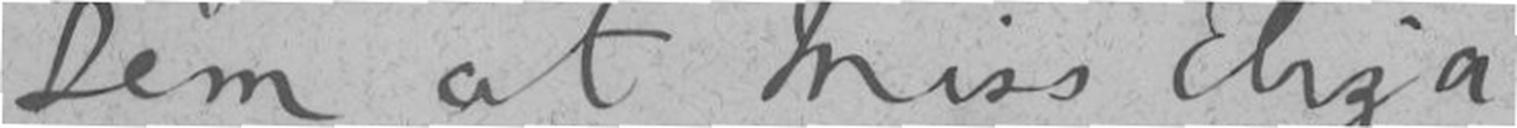Dem at Miss Eliza-
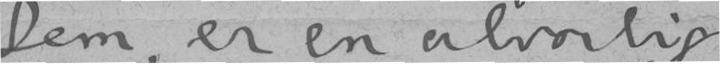Dem, er en alvorlig
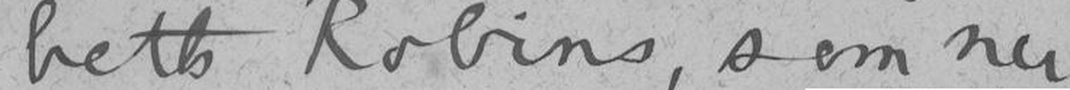beth Robins, som nu
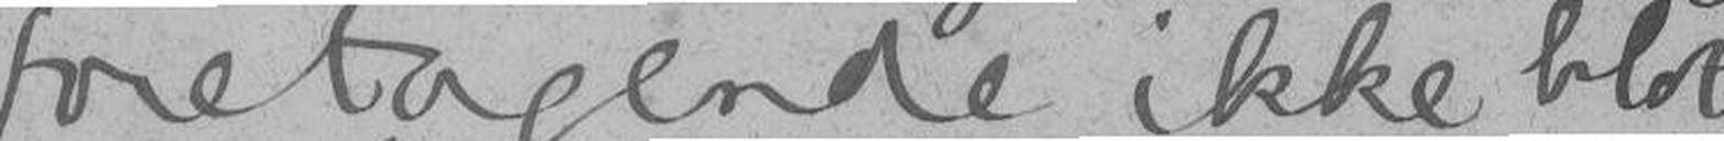foretagende ikke blot
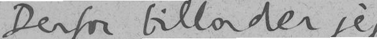Derfor tillader jeg
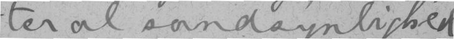ter al sandsynlighed,
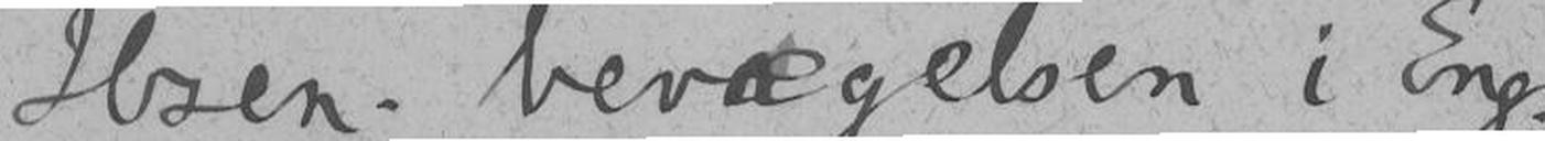Ibsen bevægelsen i Eng-
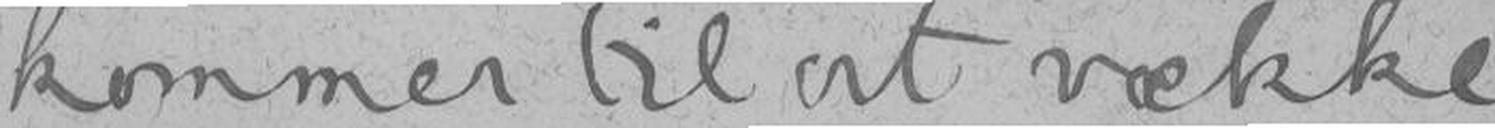kommer til at vække
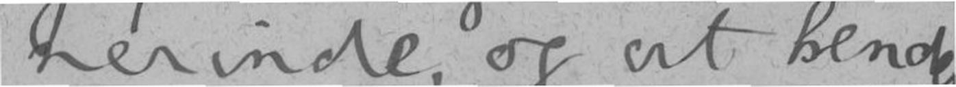nerinde, og at hendes
